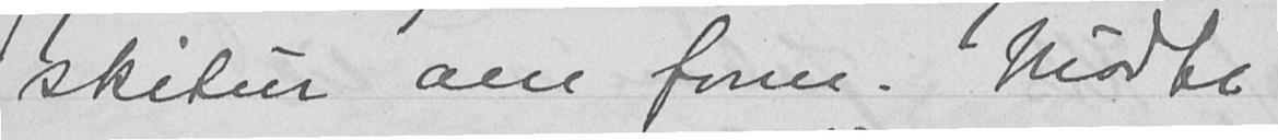skitur om form. Mødte
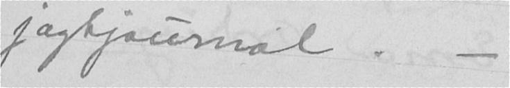jagtjournal. -
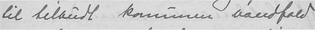til tilbudt komunen vandfald
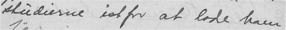studierne ist for at lade ham
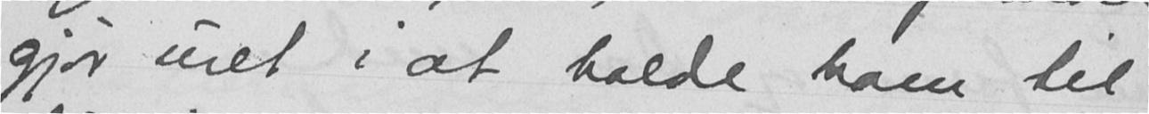gjør uret i at holde ham til
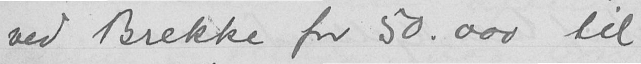ved Brekke for 50.000 til
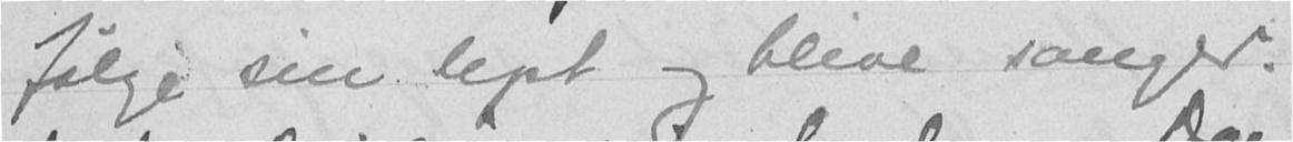følge sin lept og blive sanger.
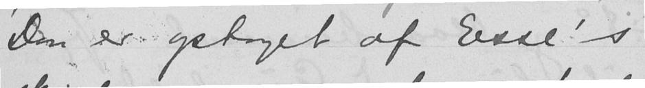Don er optaget af Esse's
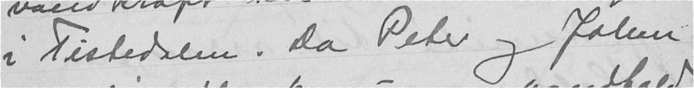i Tistedalen. Da Peter og Johan
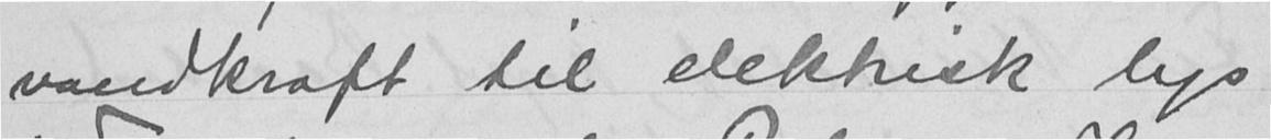vandkraft til elektrisk lys
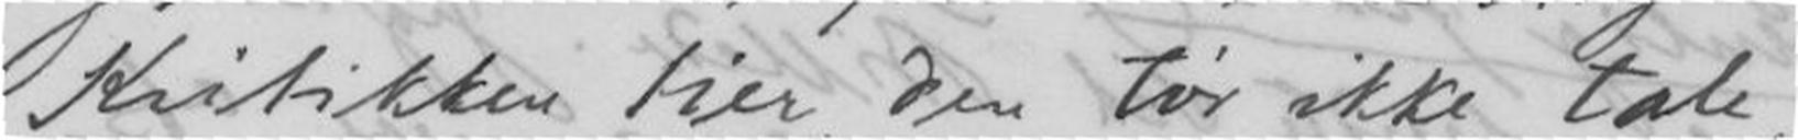Kritikken tier, den tør ikke tale,
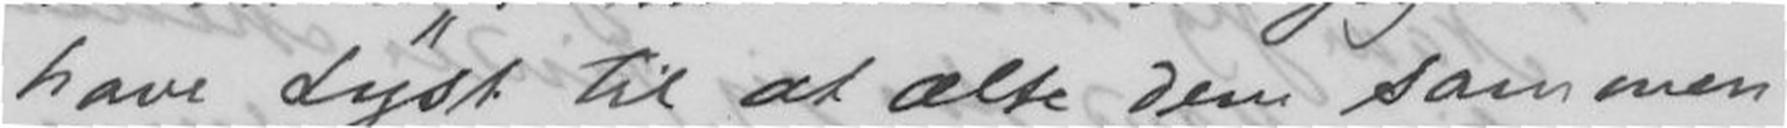have Lyst til at ælte dem sammen
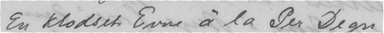En klodset Evne à la Per Degn,
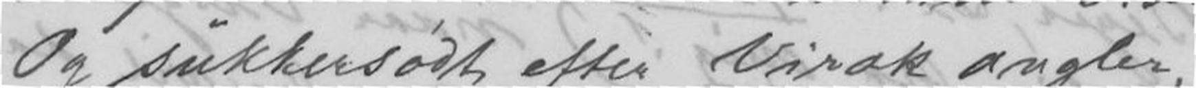Og sukkersødt efter Virak angler,
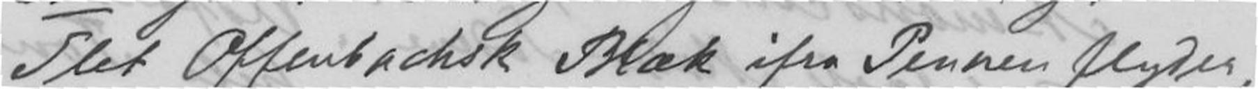Slet Offenbachsk Blæk ifra Pennen flyder,
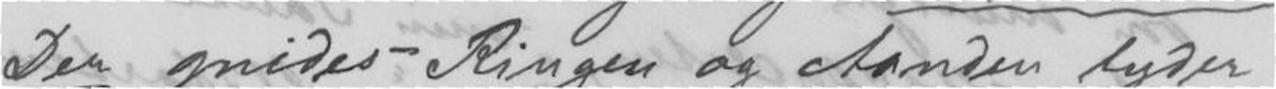Der gnides - Ringen og Aanden lyder
